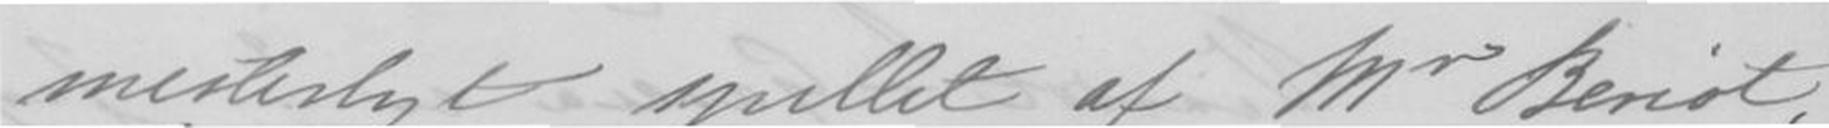mesterligt spillet af Mr Beriot,
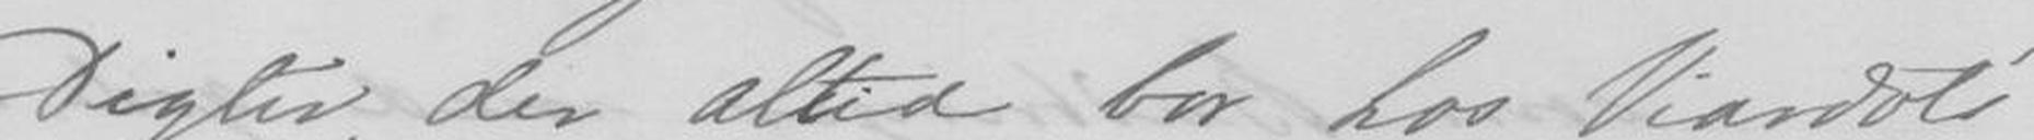Digter, der altid bor hos Viardot's
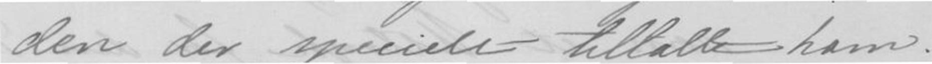den der specielt tiltrakk ham.
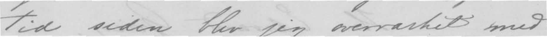Tid siden blir jeg overrasket med
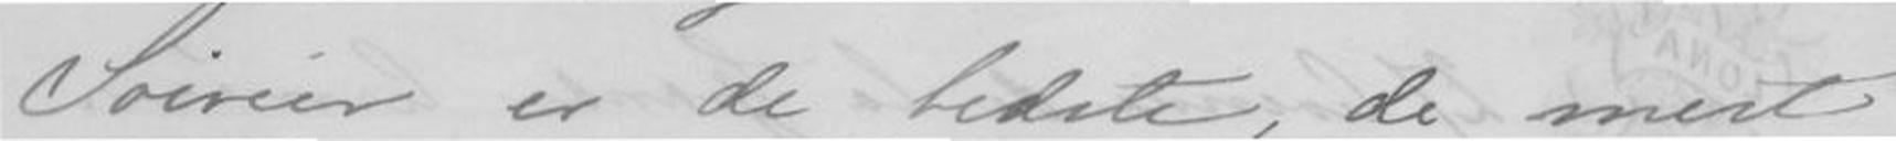Soireér er de bedste, de mest
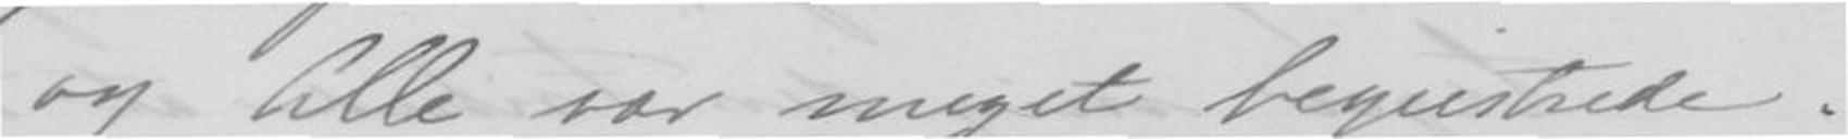og Alle var meget begeistrede.
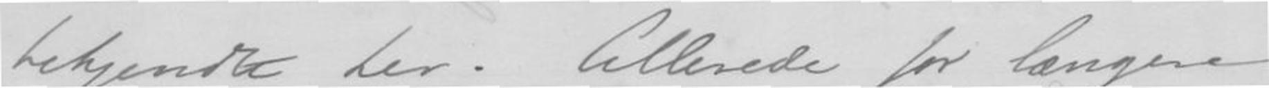bekjendte der. Allerede for længere
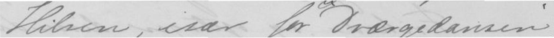Hilsen, især for Dværgedansen,
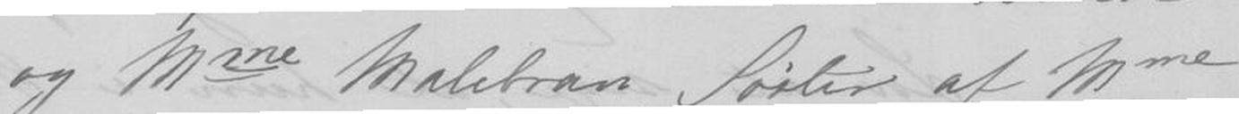og Mme Malebran, Søster af Mme
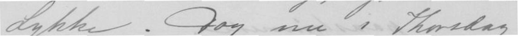Lykke. Dog nu i Thorsdag
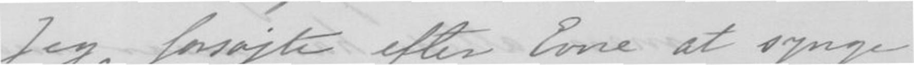Jeg forsögte efter Evne at synge
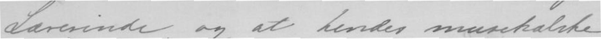Lærerinde og at hendes musikalske
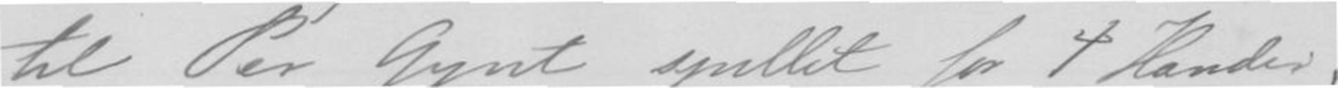til Per Gynt spillet for to Hænder,
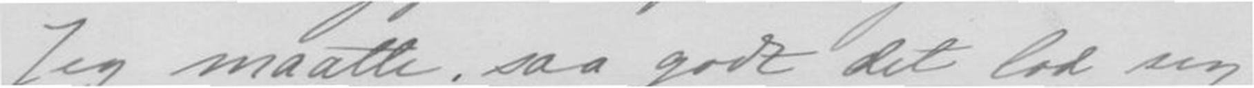Jeg maatte, saa godt det lod sig
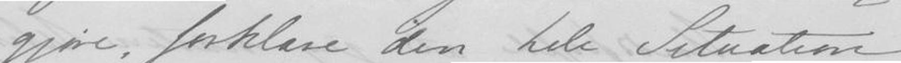gjøre, forklare den hele Situation
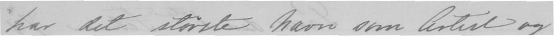har det største Navn som Artist og
